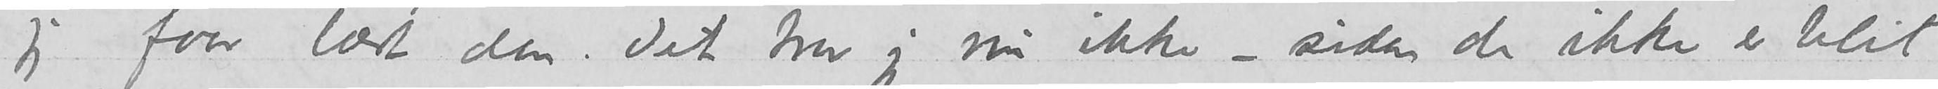jeg faar læst den.  Det tror jeg nu ikke – siden de ikke er blit
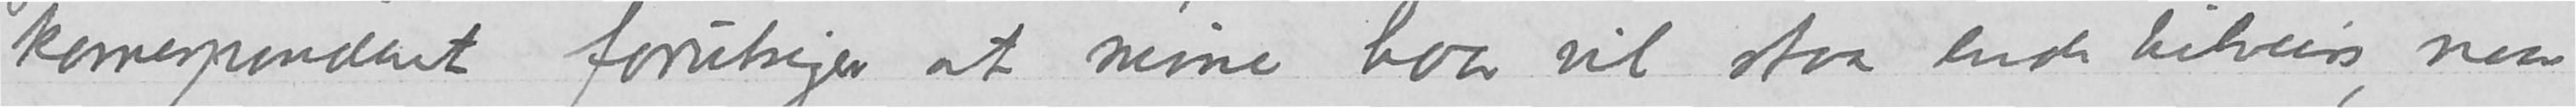korrespondent forutsiger at mine haar vil staa enda tilveirs, naar
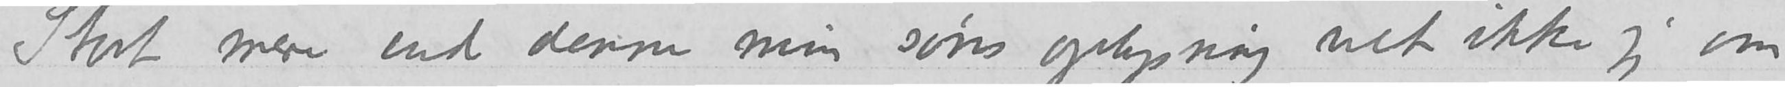Stort mere end denne min søns oplysning vet ikke jeg om
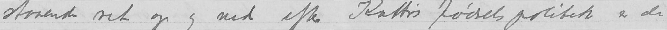staaende ret op og ned efter Kattis fødselspolitikk er de
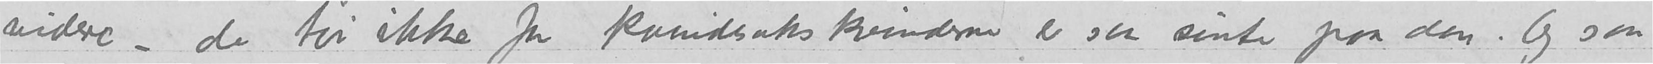videre – de tør ikke for kvindesakskvinderne er saa sinte paa den. Og saa
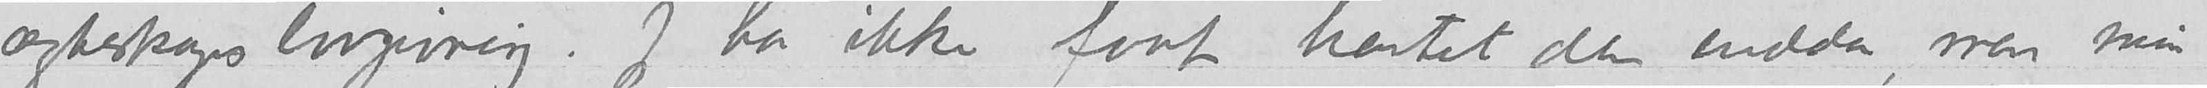ægteskapslovgivning.  Jeg har ikke faat hentet den endda, men min
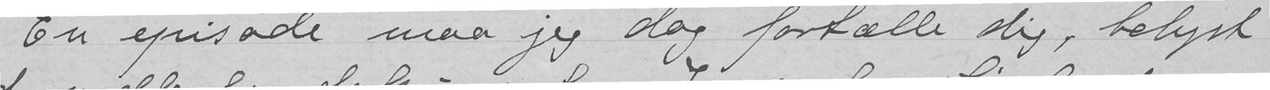En episode maa jeg dog fortælle dig, belyst
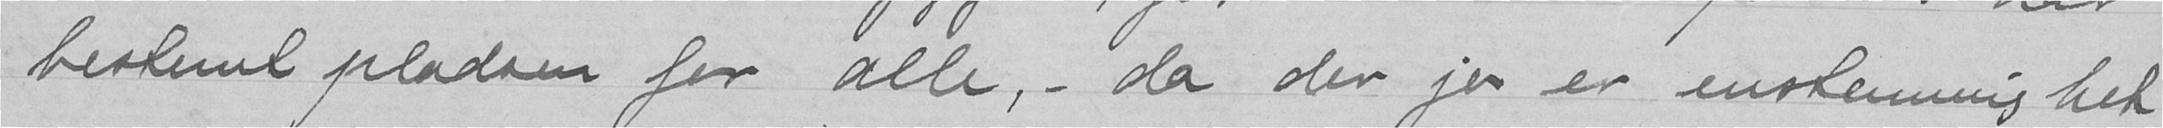bestemt pladsen for alle,- da der jo er enstemmighet
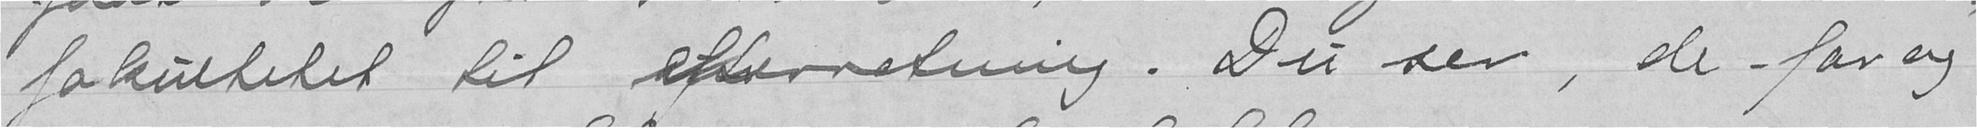fakultetet til efterretning. Du sen, de - far og
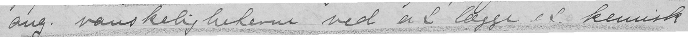ang. vanskelighederne ved at lægge et kemisk
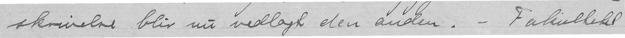skrivelse blir nu vedlagt den anden. - Fakultets
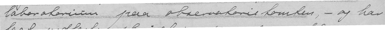laboratorim paa observatorietomten, - og har
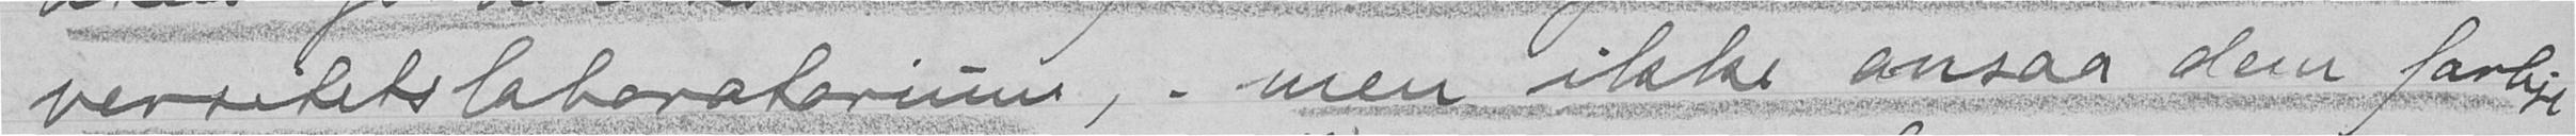versitetslaboratorium, - men itkae ansaa den farlig
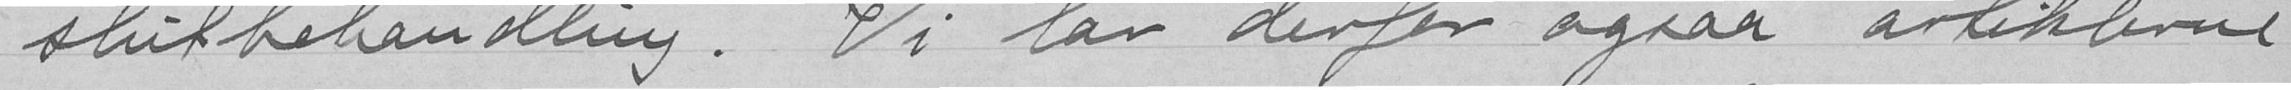slutbehandling. Vi lar derfor ogsaa artiklerne
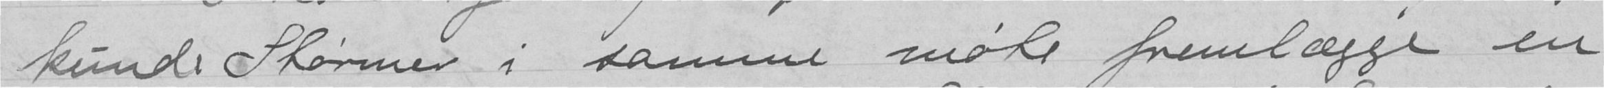kunde Størmer i samme møte fremlægge en
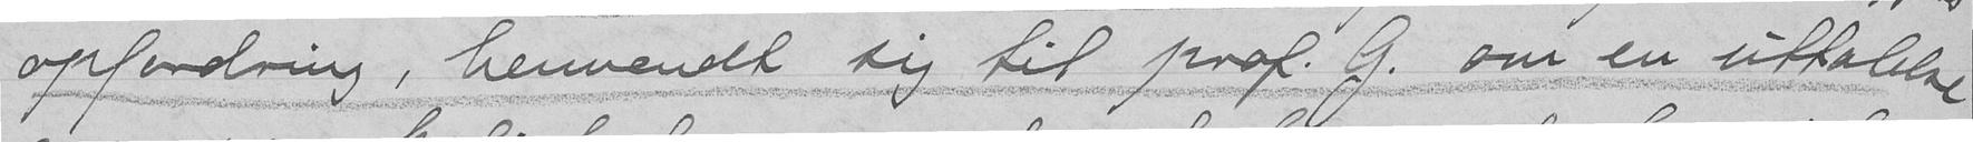opfordring, henvendt sig til prof. G. om en uttalelse
